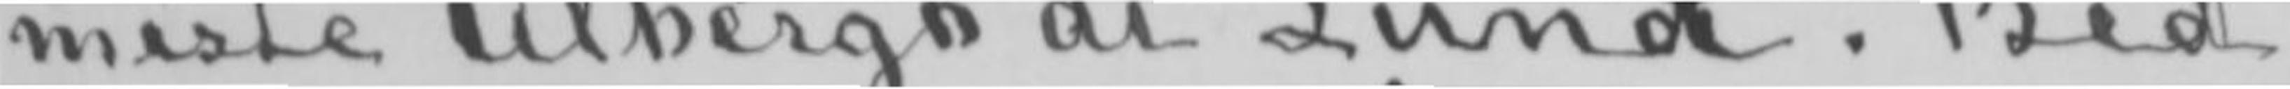meste Albergo di Luna. Bed
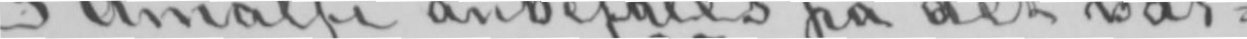I Amalfi anbefales på det var-
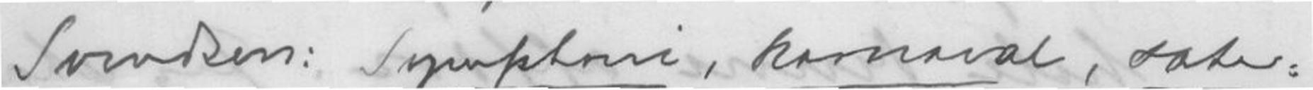Svendsen:Symphoni, karneval, sæter-
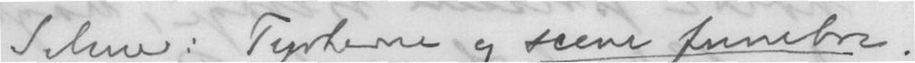Selmer:Tyrkerne og scene funebre
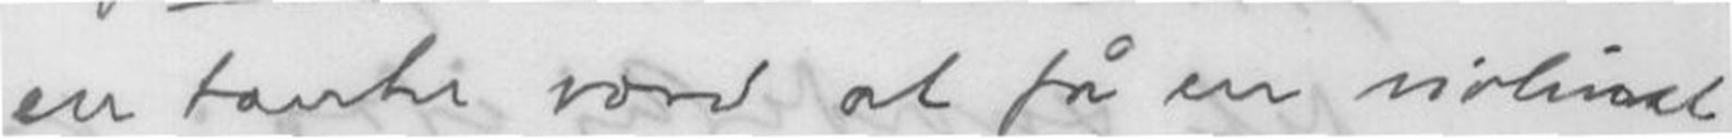en tanke værd at få en violinist
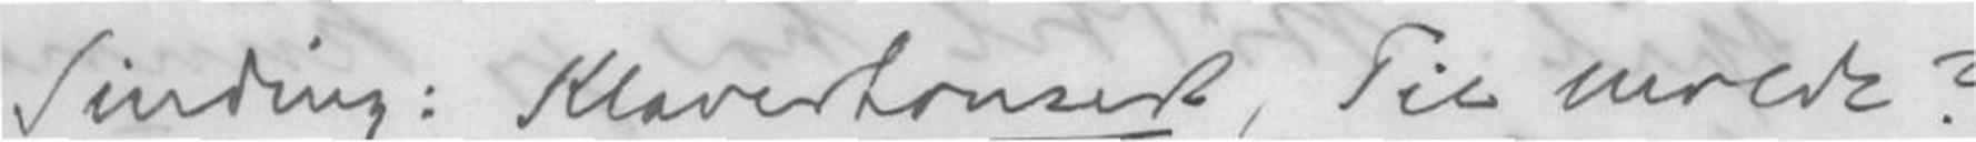Sinding:Klaverkonsert, Til Molde?
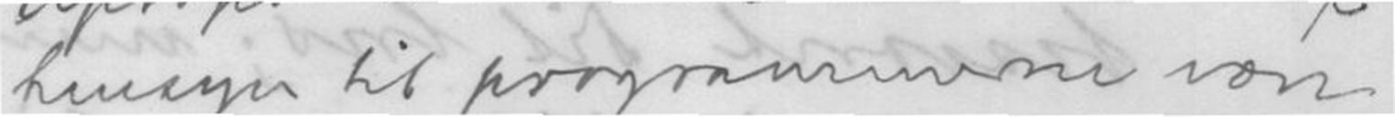hensyn til programmene være
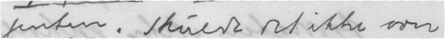jenten. Skulde det ikke være
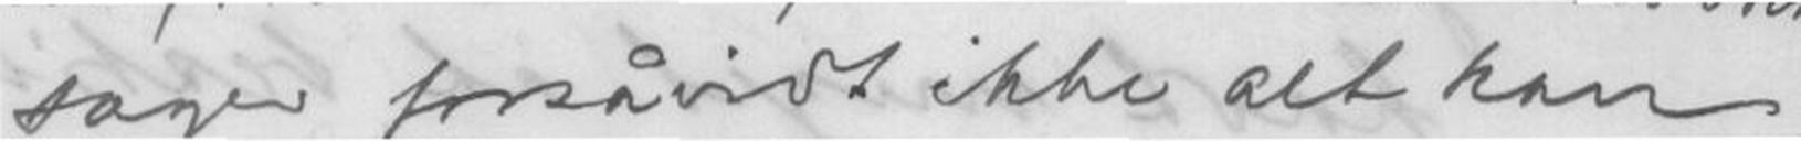sager forsåvidt ikke alt kan
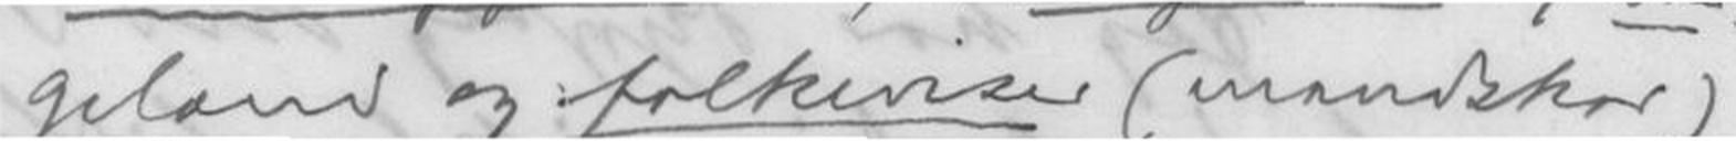geland og folkeviser (mandskor)
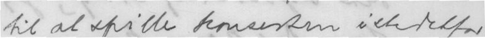til at spille konserten istedetfor
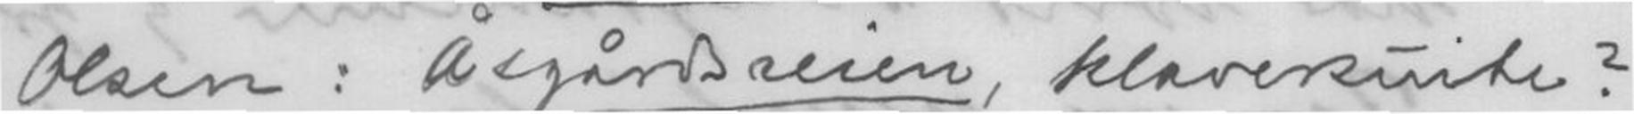Olsen:Åsgårdsreien, klaversuite?
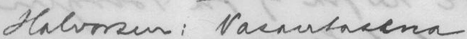Halvorsen:Vasantasena
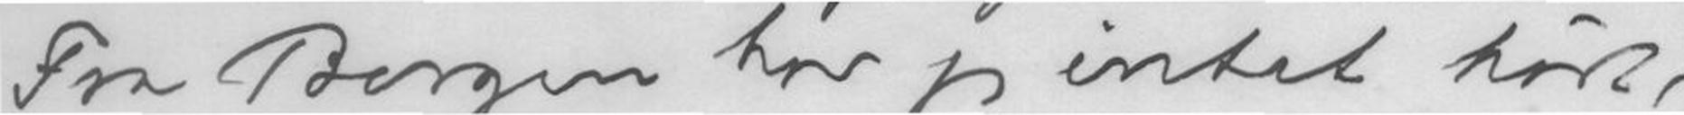Fra Bergen har jeg intet hørt,
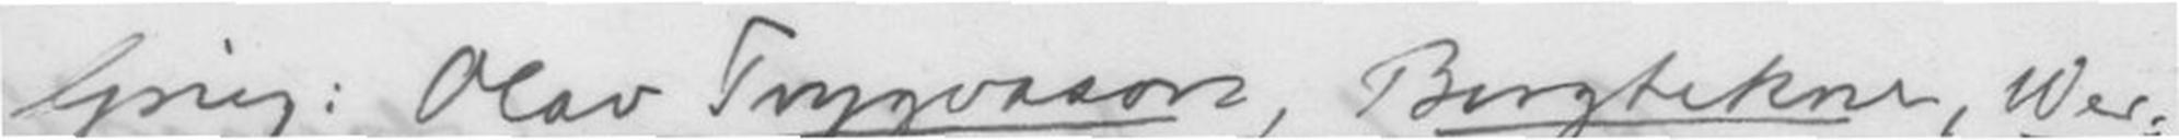Grieg:Olav Trygvason, Bergtegne, Wer-
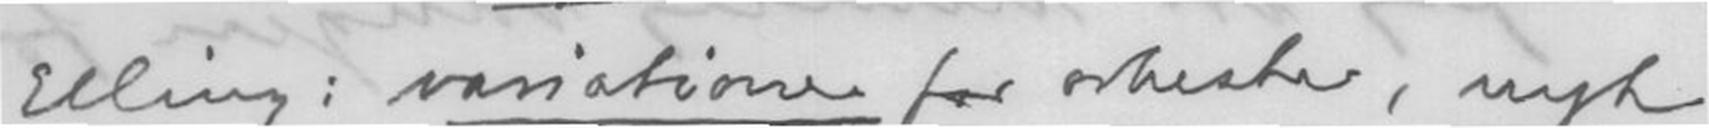Elling:Variationer for orkester, nyt
